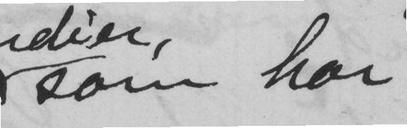som har
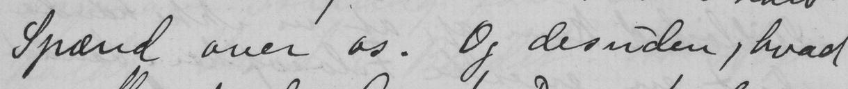Spænd over os. Og desuden, hvad
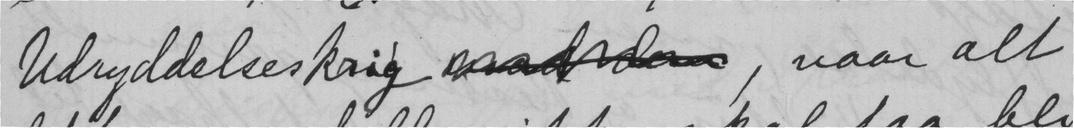Udryddelseskrig , naar alt
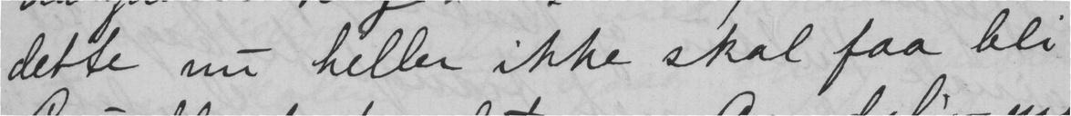dette nu heller ikke skal faa bli
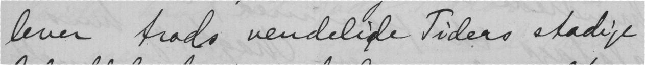lever trods uendelide Tiders stadige
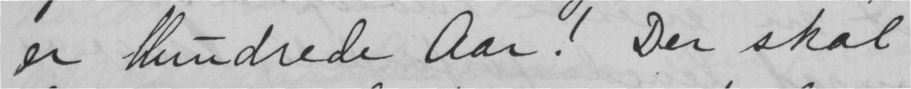er Hundrede aar! Der skal
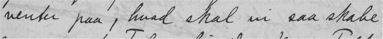venter paa, hvad skal vi saa skabe
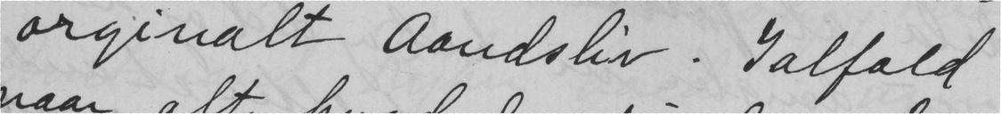originalt Aandsliv. I alfald
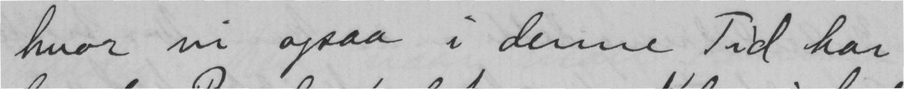hvor vi ogsaa i denne Tid har
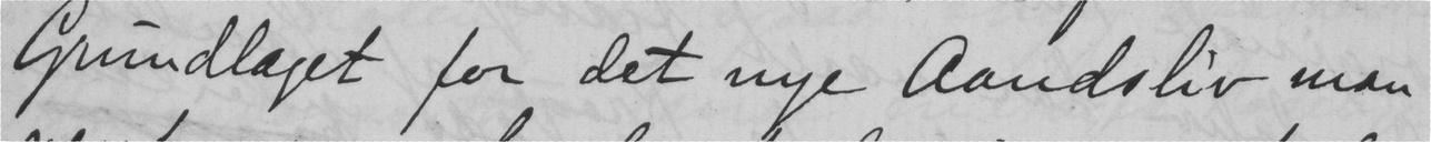Grundlaget for det nye Aandsliv man
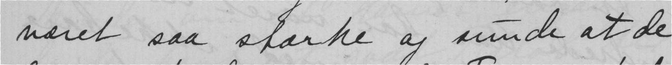været saa stærke og sunde at de
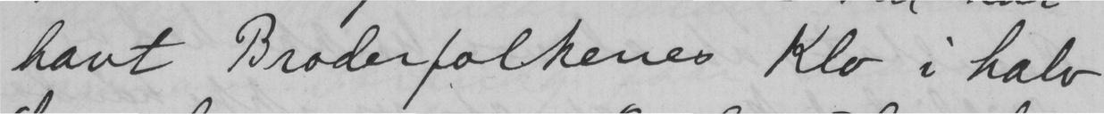havt Broderfolkenes Klo i halv
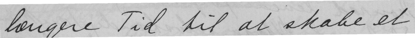længere Tid til at skabe et
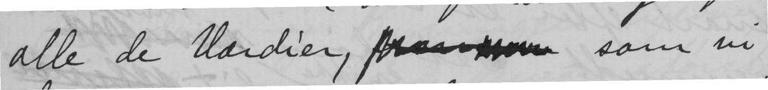alle de Værdier,  som vi
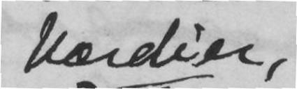værdier
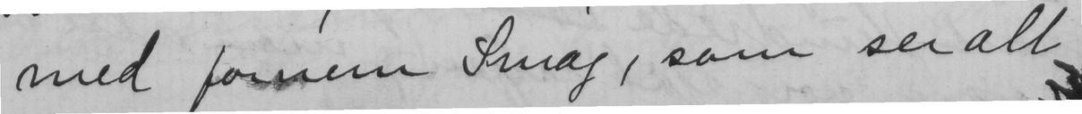med fornem Smag, som ser alt
